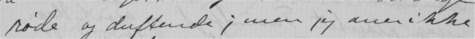røde og duftende; men jeg aner ikke
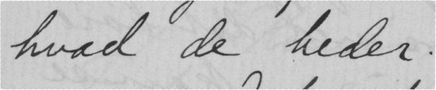hvad de heder.
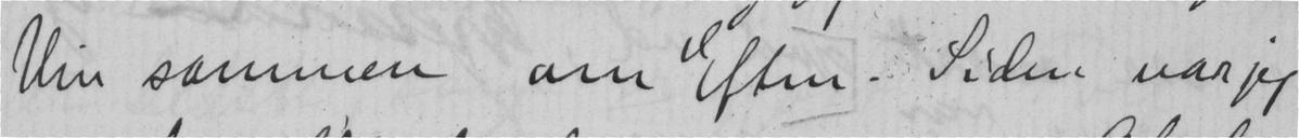Vin sammen om Eftm. Siden var jeg
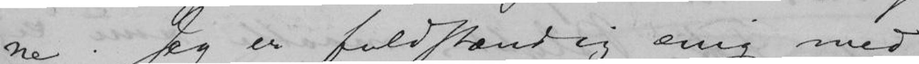ne. Jeg er fuldstændig enig med
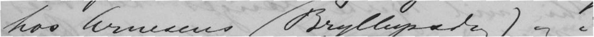hos Arnesens (Bryllupsdag) og i
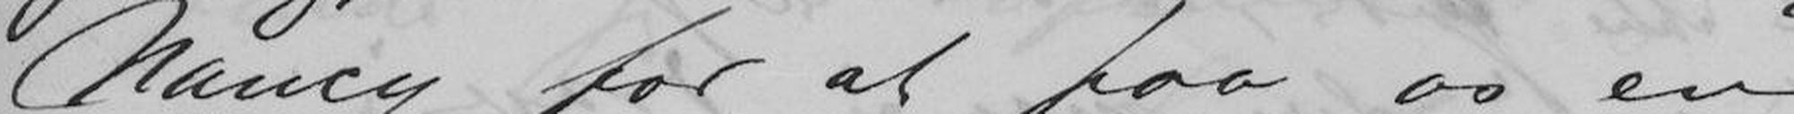Nancy for at faa os en
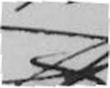F
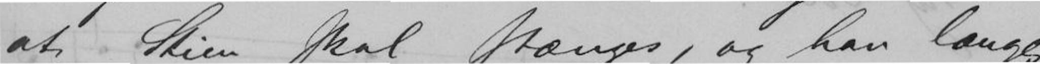at Stien skal stænges, og han længes
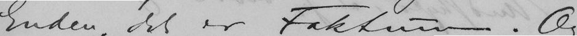Enden, det er Faktum. Og
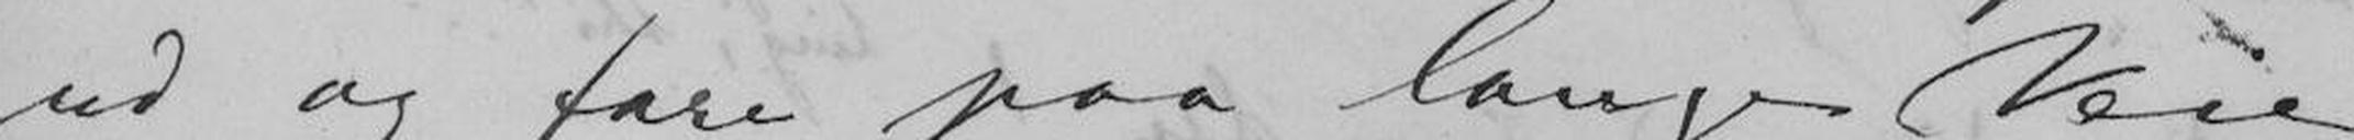ud og fare paa lange Veie.
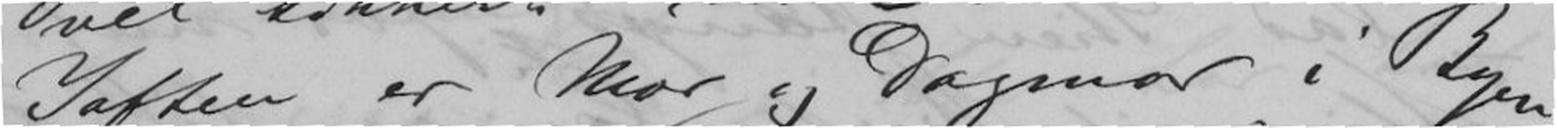Iaften er Mor og Dagmar i Byen
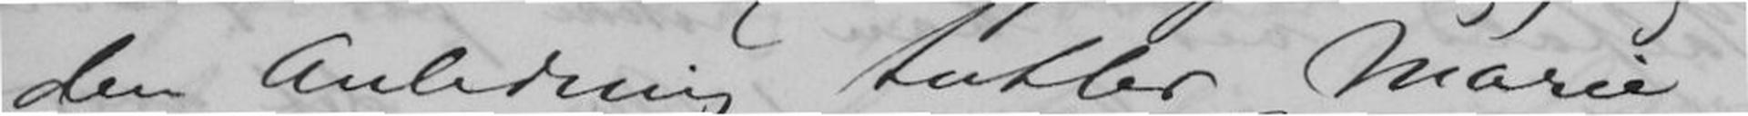den Anledning tutler Marie og
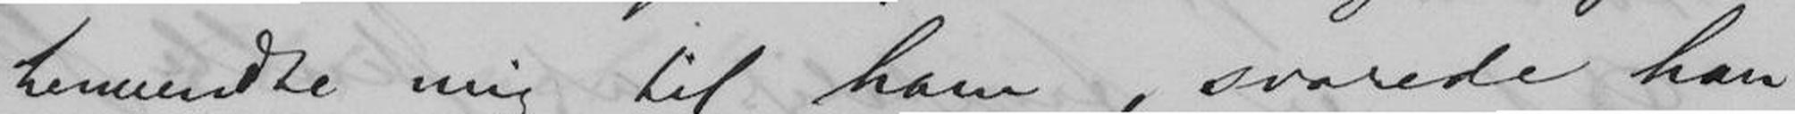henvendte mig til ham, svarede han
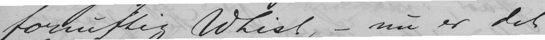fornuftig Whist, - nu er det
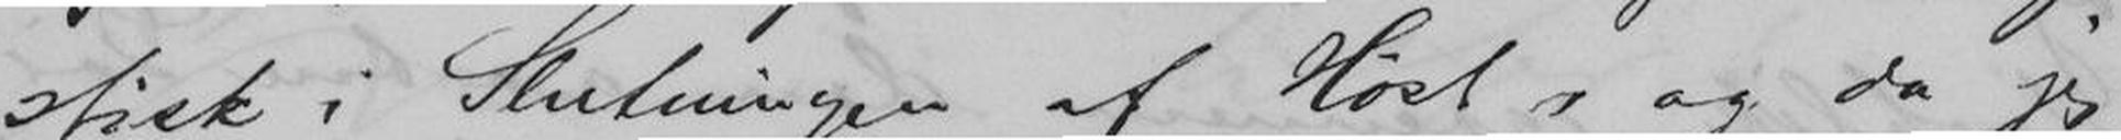stisk i Slutningen af Høst og da jeg
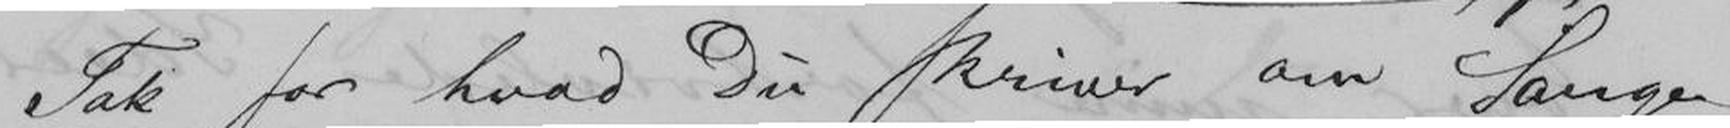Tak for hvad Du skriver om Sange-
